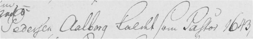Pedersen Aalborg kaldet som Pastor 1643,
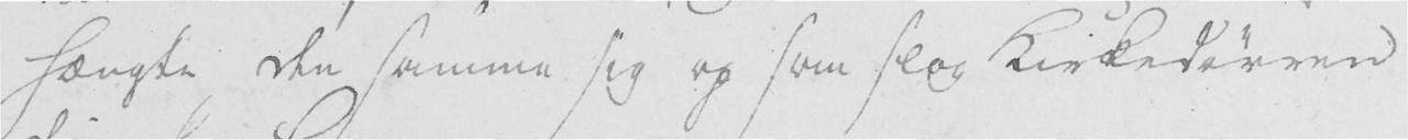hængte den samme sig op som slog Kirkedørren
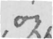og
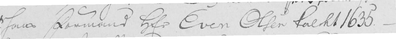hans Formand Hr Even Olsen kaldet 1635. -
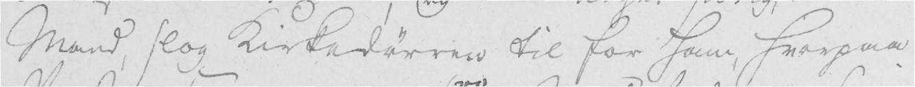Mand, slog Kirkedørren til for ham, hvorpaa
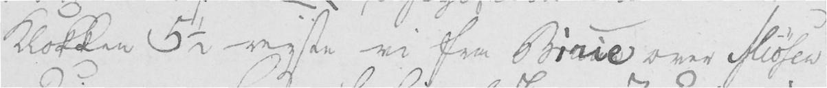Klokken 5 1/2 reyste vi fra Birie over Miøsen
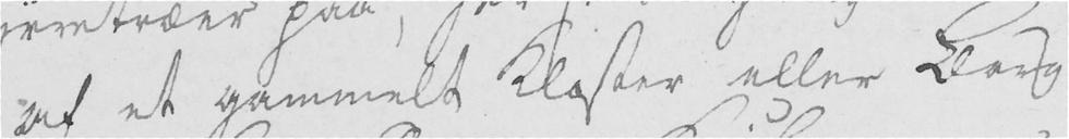af et gammelt Kloster eller Borg
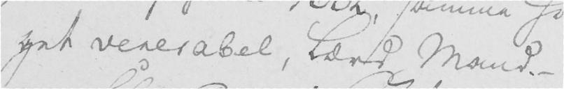get venerabel, lærd Mand. -
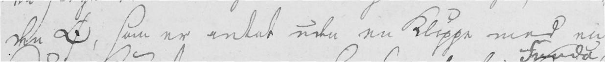den Ø, som er intet uden en Klippe med en
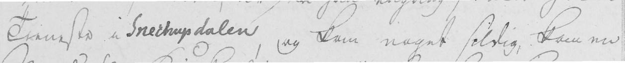Tieneste i Snertingsdalen, og kom noget sildig, kom en
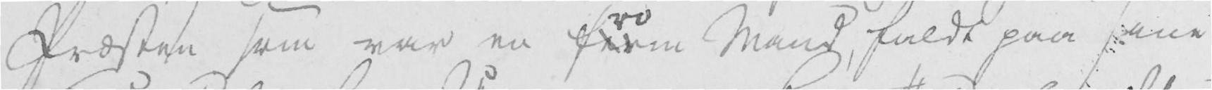Præsten som var en from Mand, faldt paa sine
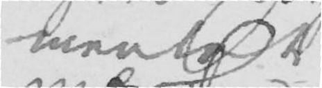mueligt
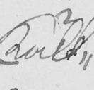kalt
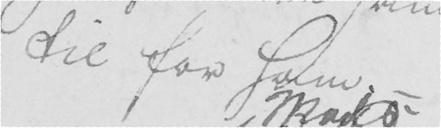til for ham. -
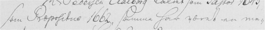som Propositus 1662, samme har været en me-
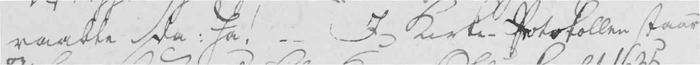raabte da: Ja! - - I Kirke-Protokollen staar
